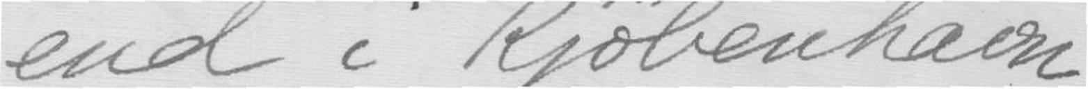end i Kjøbenhavn
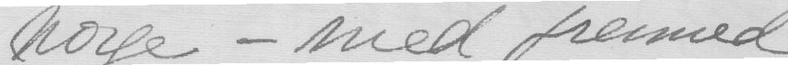Norge - med fremmed
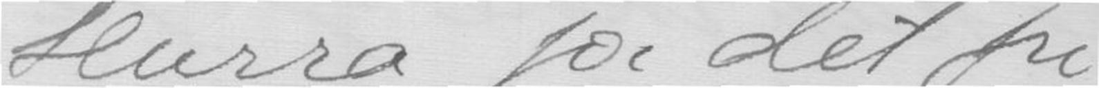Hurra for det fri
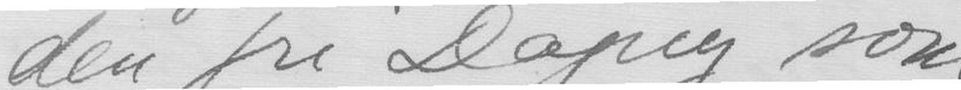den fri Dagny som
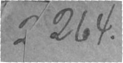264.
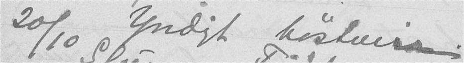20/10 Yndigt høstveir.
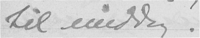til middag.
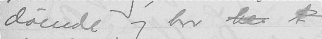døende og har her t
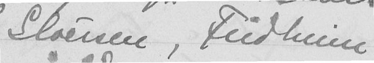Gløersen, Fridheim
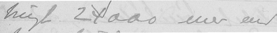brugt 24000 mer end
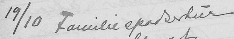19/10 Familiespadsertur
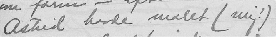Astrid havde malet (mig!)
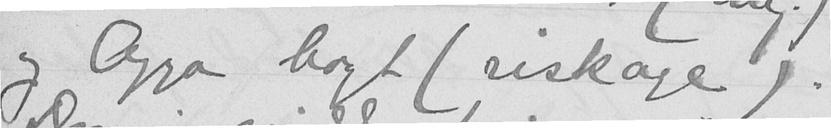og Agga bagt (riskage).
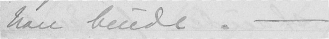han burde. -
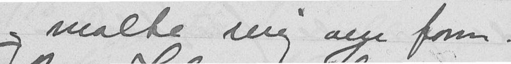og malte mig om form.
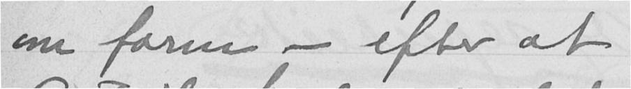om form - efter at
